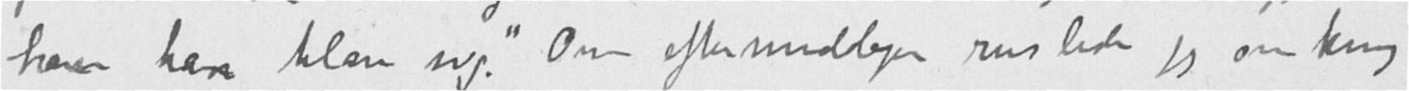han kan klare sig." Om eftermiddagen ruslede jeg omkring
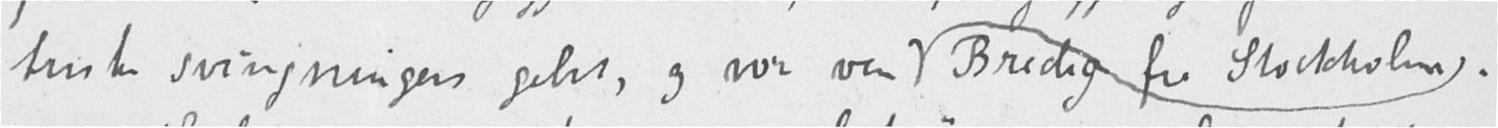triske svingningers gebet, og vor ven Bredig fra Stockholm.
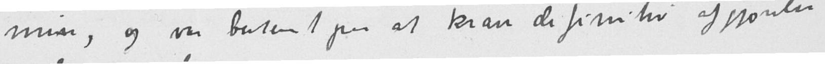min, og var bestemt paa at kræve definitiv afgjørelse
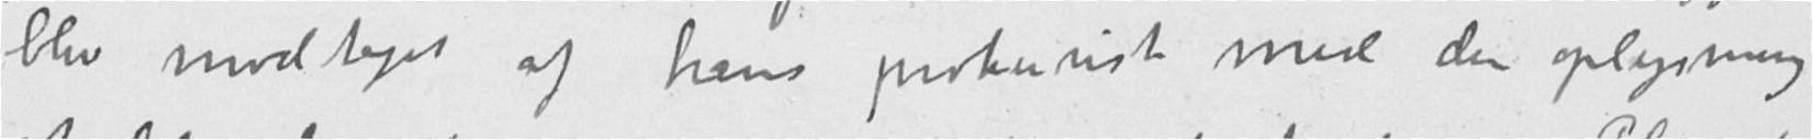blev modtaget af hans prokuriste med den oplysning
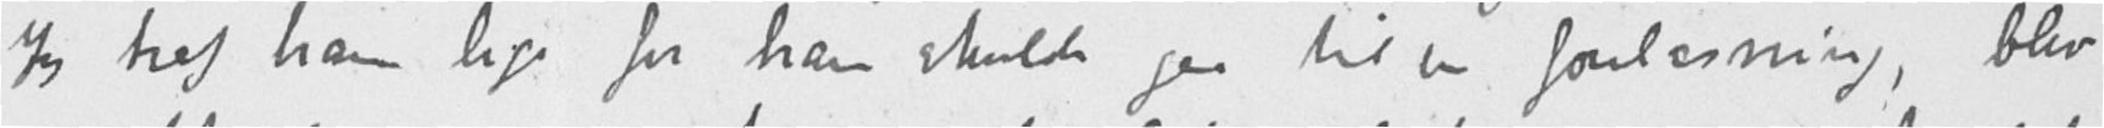Jeg traf ham lige før han skulde gaa til en forelæsning, blev
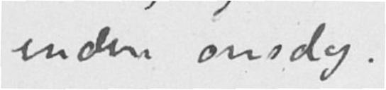inden onsdag.
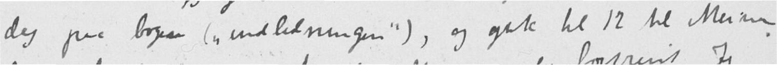dag paa bogen ("indledningen"), og gik kl 12 til Meiner
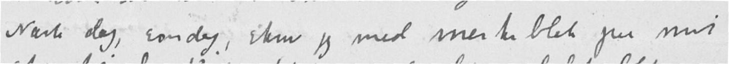Næste dag, søndag, skrev jeg med merkeblæk paa mit
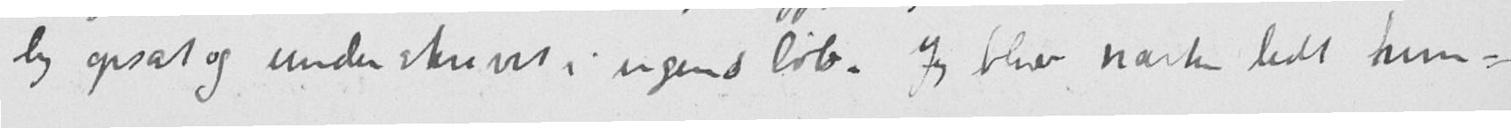lig opsat og underskrevet i ugens løb. Jeg blev næsten lidt him-
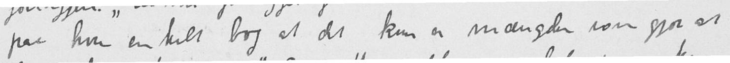paa hver enkelt bog at det kun er mængden som gjør at
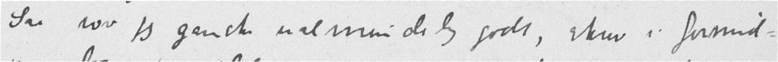Saa sov jeg ganske ualmindelig godt, skrev i formid-
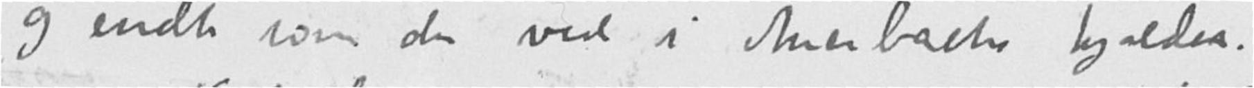og endte som du ved i Auerbachs kjælder.
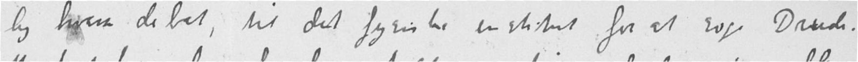lig hissige debat, til det fysiske institut for at søge Druda.
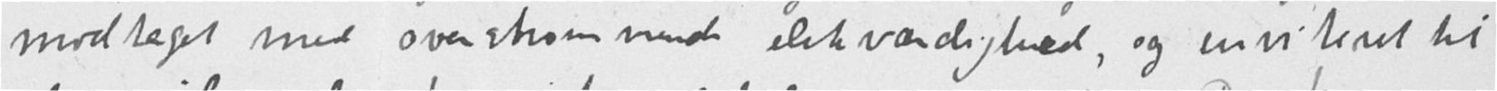modtaget med overstrømmende elskværdighed, og inviteret til
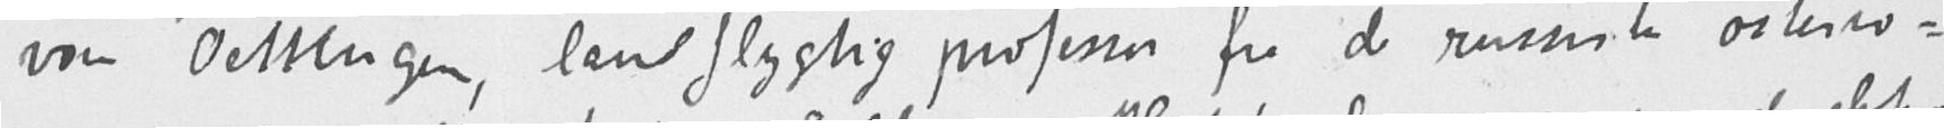von Oettingen, landflygtig professer fra de russiske østersø-
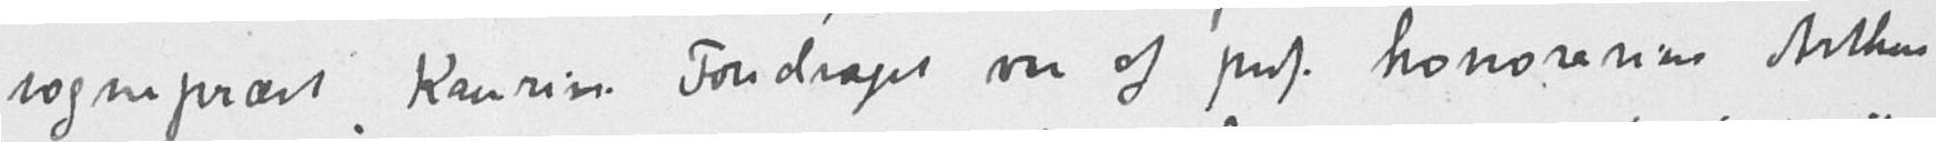sognepræst Kaurin. Foredraget var af prof. honorarius Arthur
